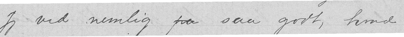Jeg ved nemlig fra saa godt, hvad
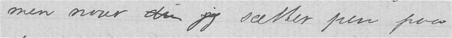men naar du jeg sætter pen paa
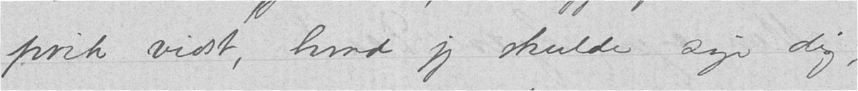prik vidst, hvad jeg skulde sige dig,
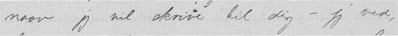naar jeg vil skrive til dig - jeg ved,
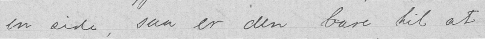en side, saa er den bare til at
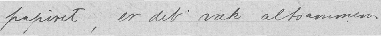papiret, er det væk altsammen.
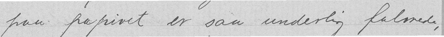paa papiret er saa underlig falmede,
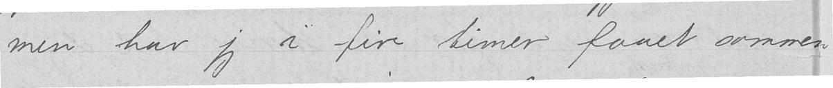men har jeg i fire timer faaet sammen
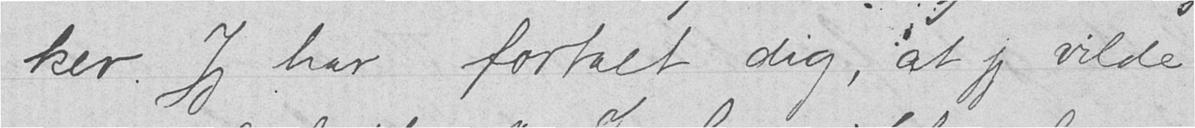ker. Jeg har fortalt dig, at jeg vilde
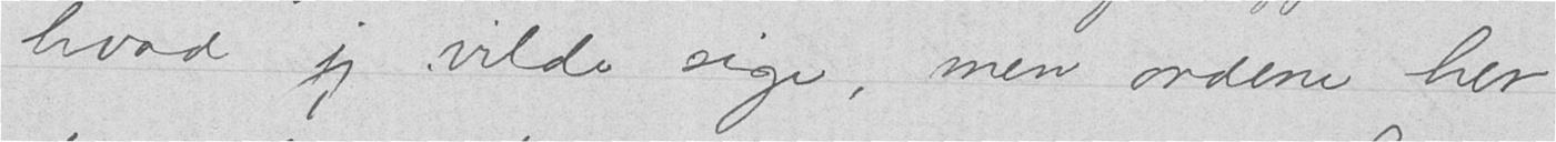hvad jeg vilde sige, men ordene her
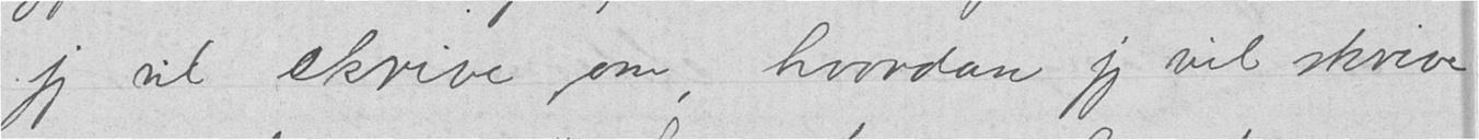jeg vil skrive om, hvordan jeg vil skrive
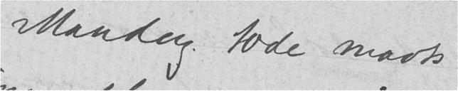Mandag 10de marts.
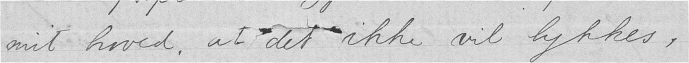mit hoved at det ikke vil lykkes.
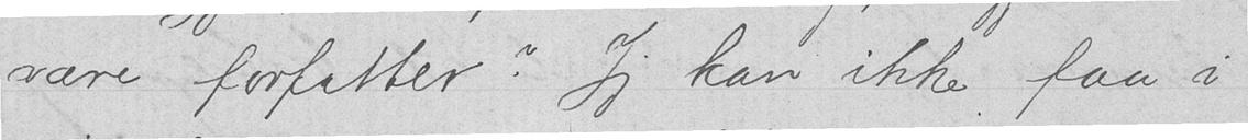være forfatter? Jeg kan ikke faa i
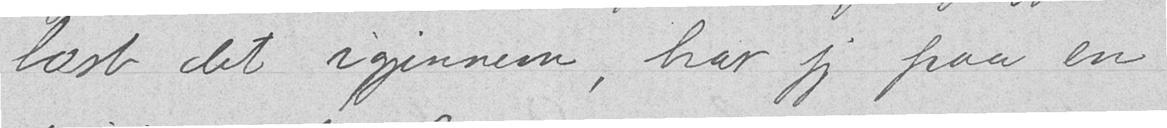læst det igjennem, har jeg paa en
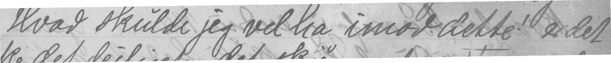Hvad skulde jeg vel ha imod dette! er det
